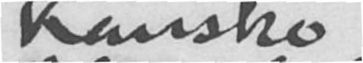Kanske
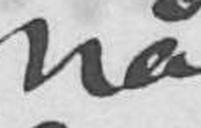når
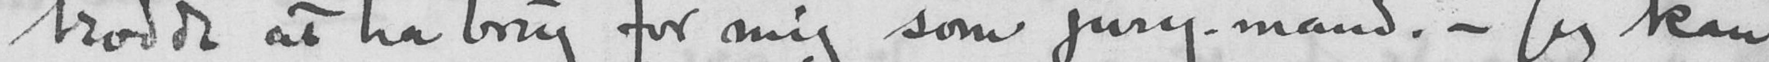trodde at ha brug for mig som jury-mand. - Jeg kan
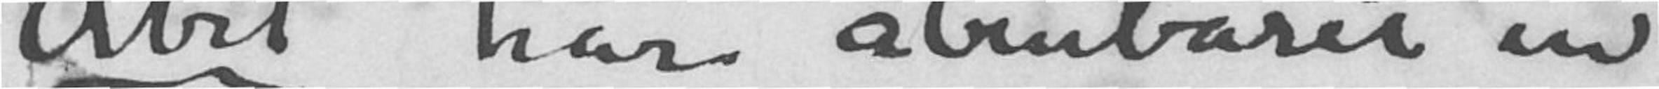"Abel" har åbenbaret en
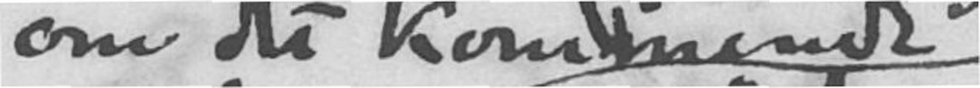om det kommende
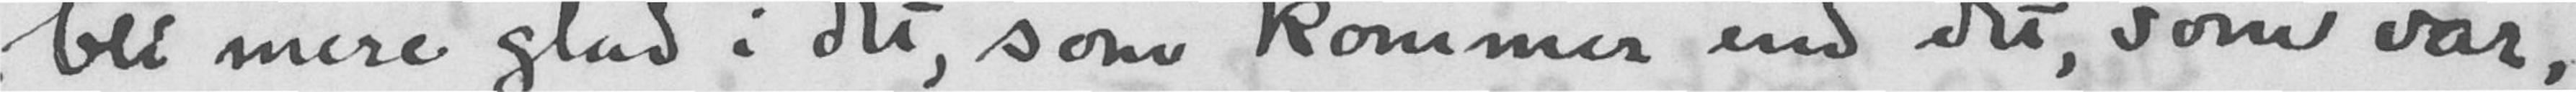bli mere glad i det, som kommer end det, som var,
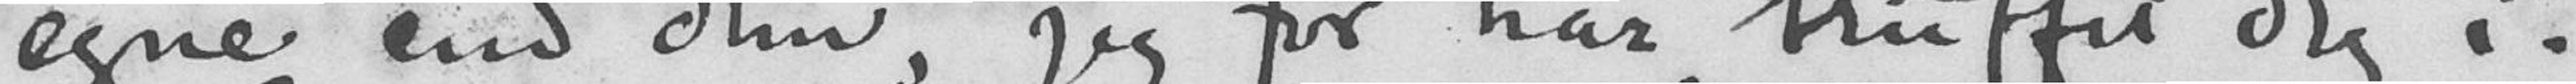egne end dem, jeg før har truffet dig i.
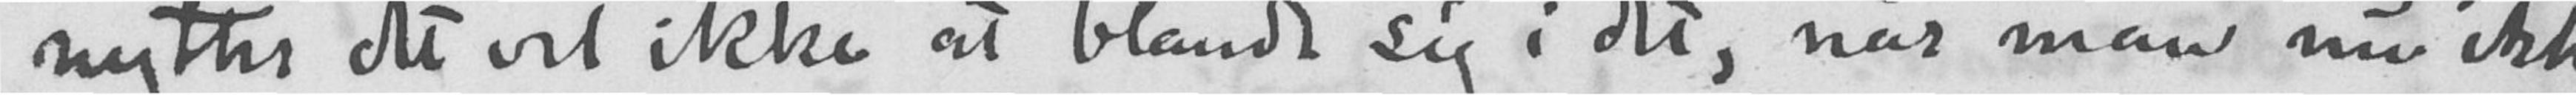nytter det vel ikke at blande sig i det, når man nu ikke
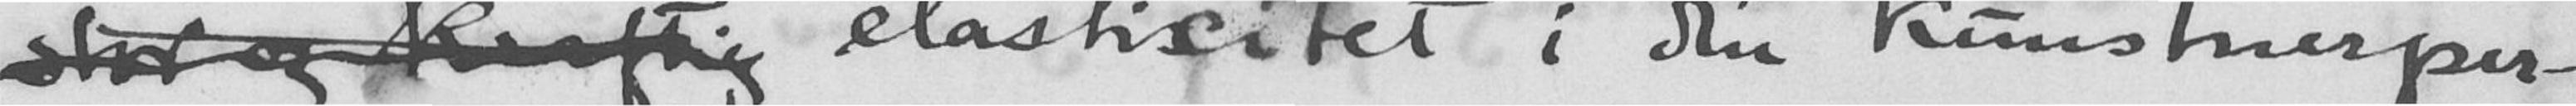stor og kraftig elasticitet i din kunstnerper-
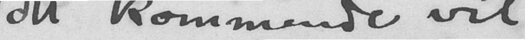det kommende vil
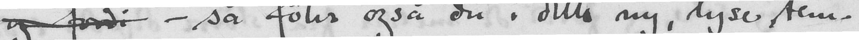og fordi - så føler også du i dette ny, lyse tem-
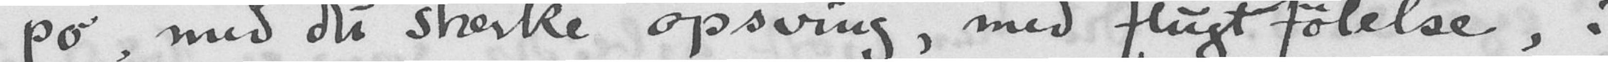po, med det stærke opsving, med flugtfølelse, i
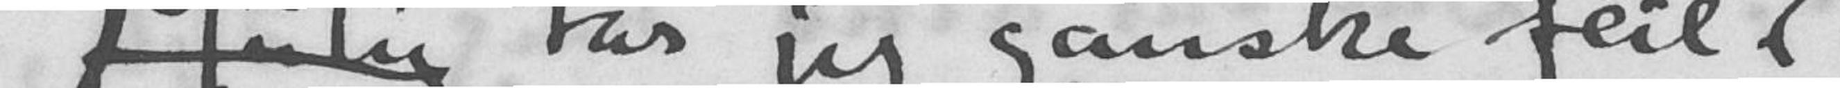Mulig tar jeg ganske feil
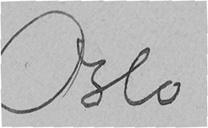Oslo
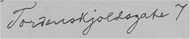Tordenskjoldsgate 7
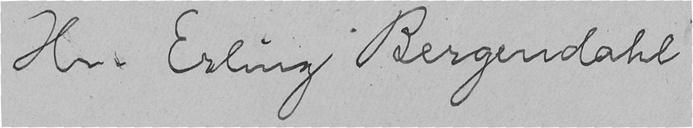Hr. Erling Bergendahl
